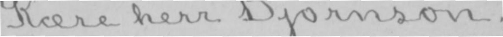Kære herr Bjørnson,
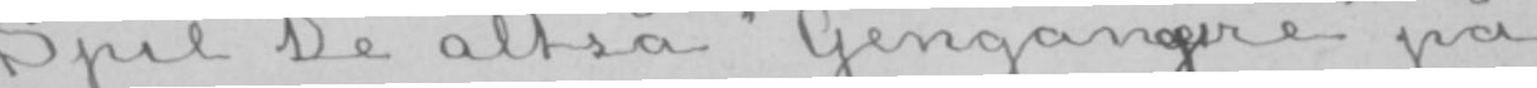Spil De altså «Genganegere» på
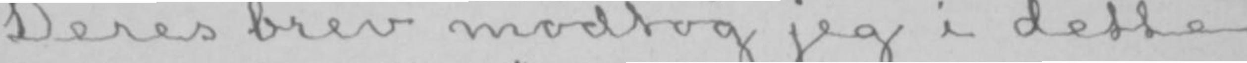Deres brev modtog jeg i dette
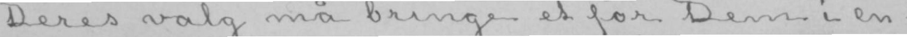Deres valg må bringe et for Dem i en-
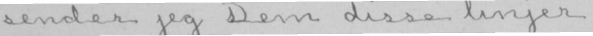sender jeg Dem disse linjer.
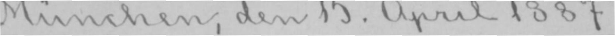München, den 15. April 1887.
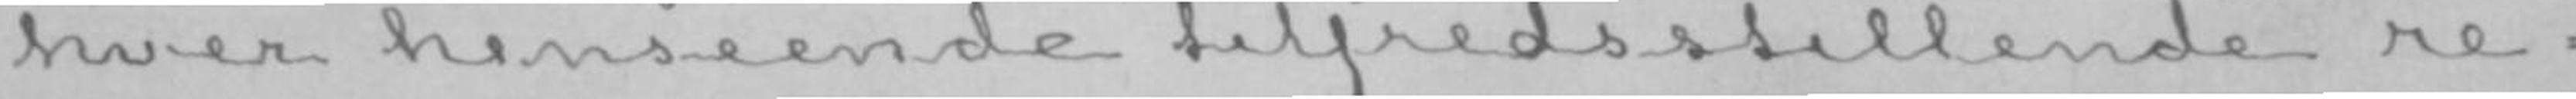hver henseende tilfredsstillende re-
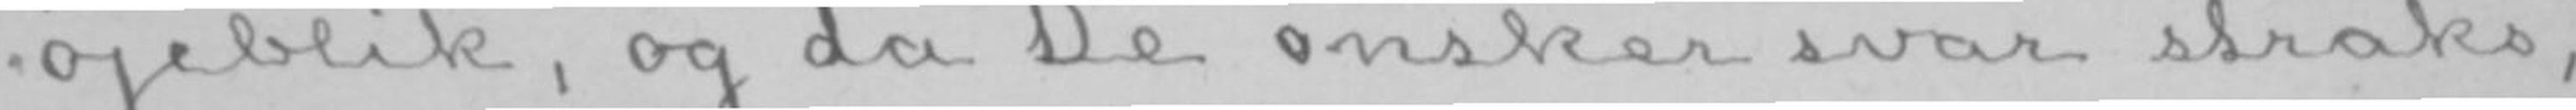øjeblik, og da De ønsker svar straks,
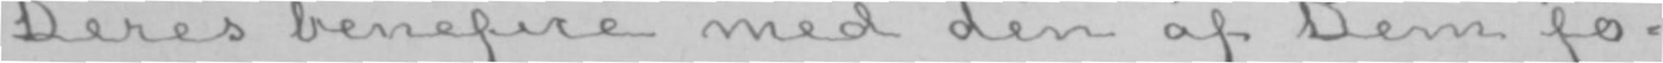Deres benefice med den af Dem fo-
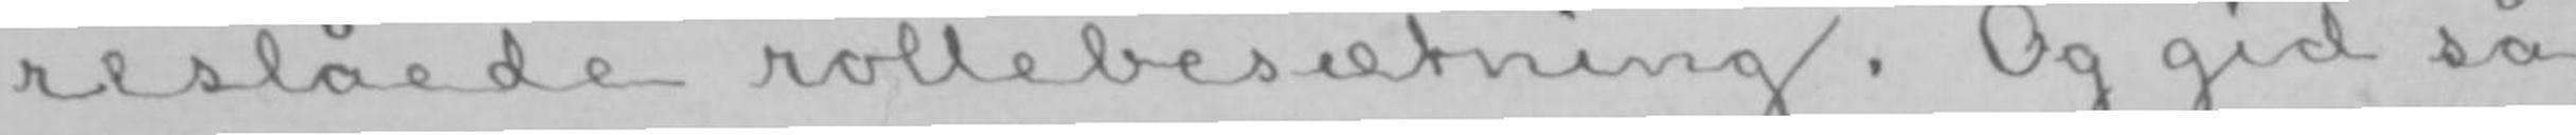reslåede rollebesætning. Og gid så
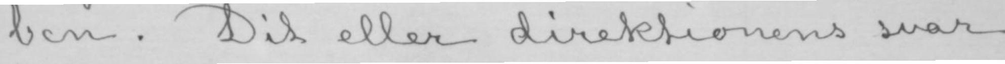ben. Dit eller direktionens svar
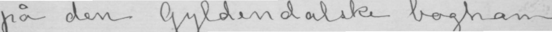på den Gyldendalske boghan-
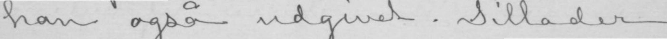han også udgivet. Tillader
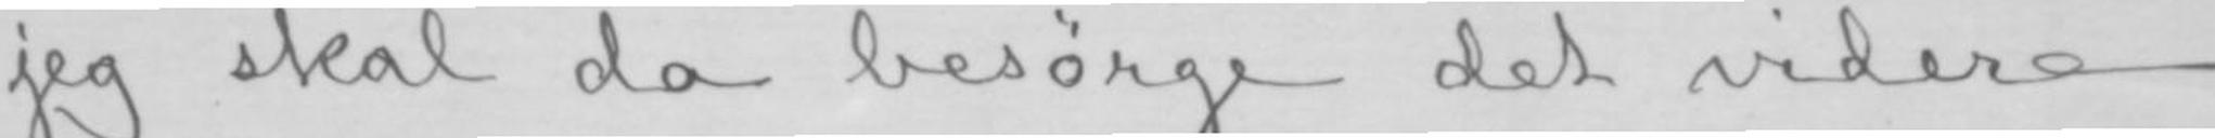jeg skal da besørge det videre.
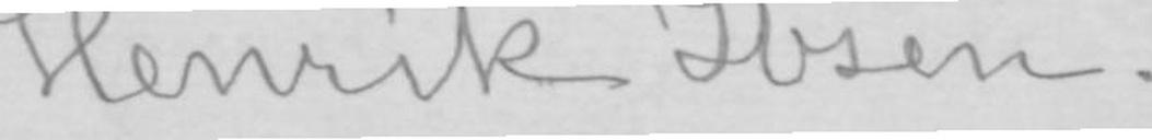Henrik Ibsen.
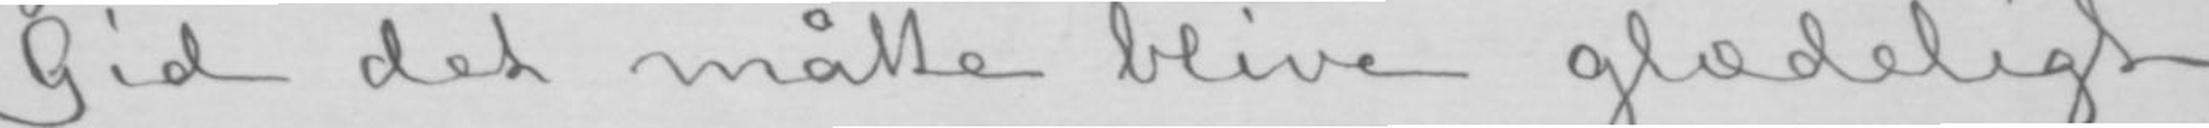Gid det måtte blive glædeligt
